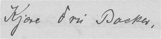Kjære Fru Backer,
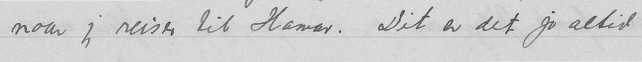naar jeg reiser til Hamar. Dit er det jo altid
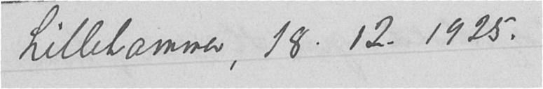Lillehammer, 18.121925.
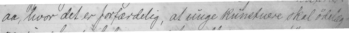aa, hvor det er forfærdelig, at unge kunstnere skal ødelæg-
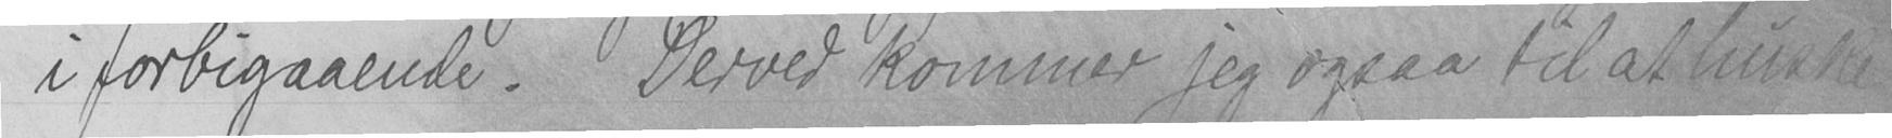i forbigaaende. Derved kommer jeg ogsaa til at huske
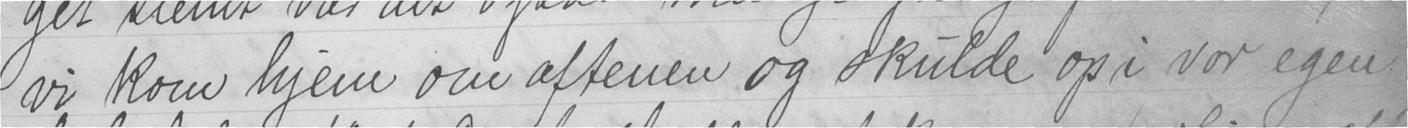vi kom hjem om aftenen og skulde op i vor egen
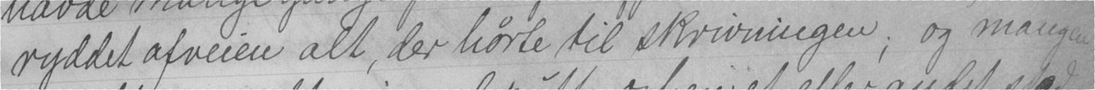ryddet afveien alt, der hørte til skrivningen; og mangen
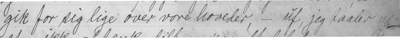gik for sig lige over vore hoveder, - uf, jeg taaler næ-
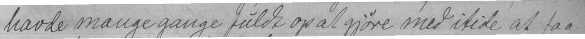havde mange gange fuldt op at gjøre med itide at faa
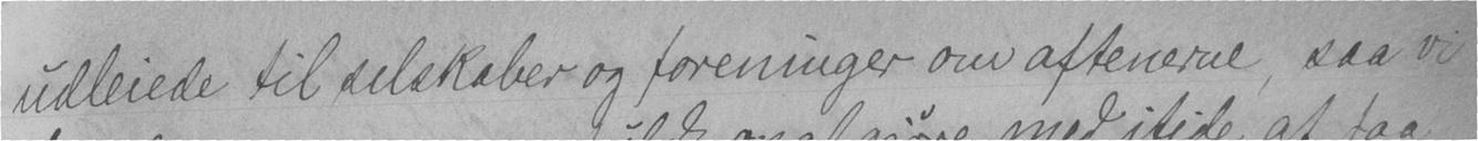udleiede til selskaber og foreninger om aftenerne, saa vi
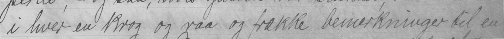i hver en krog og raa og frække bemerkninger til en
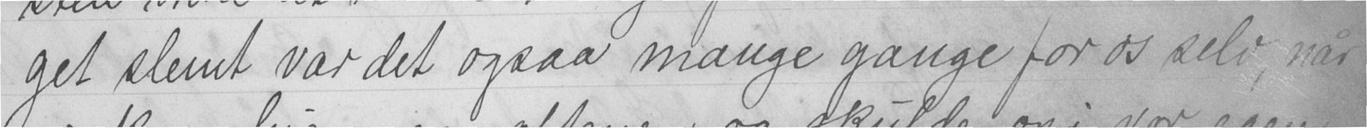get slemt var det ogsaa mange gange for os selv, når
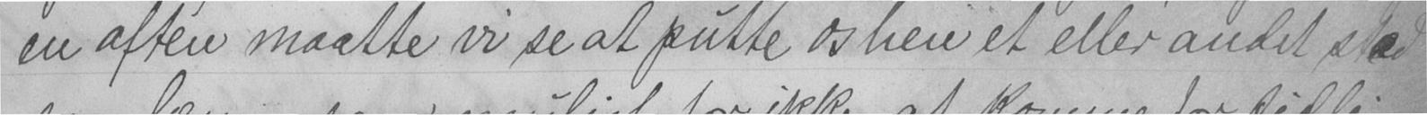en aften maatte vi se at putte os hen et eller andet stæd
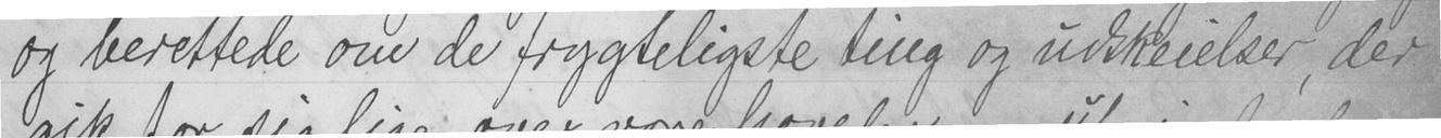og berettede om de frygteligste ting og udskeielser, der
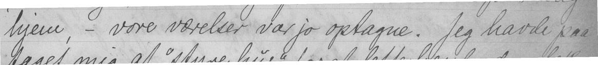hjem, - vore værelser var jo optagne. Jeg havde paa-
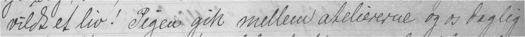vildt et liv! Pigen gik mellem ateliererne og os daglig
